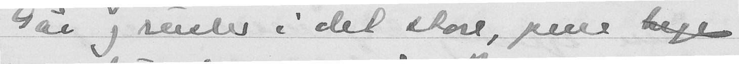Går og rusler i det store, pene
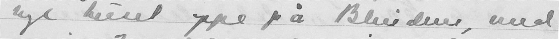nye huset oppe på Blindern, med
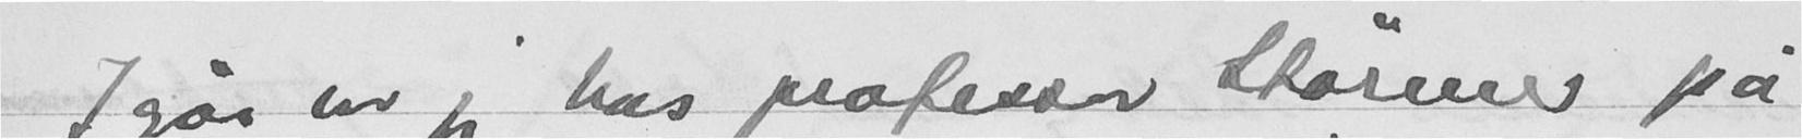Igår var jeg hos professor Størmers på
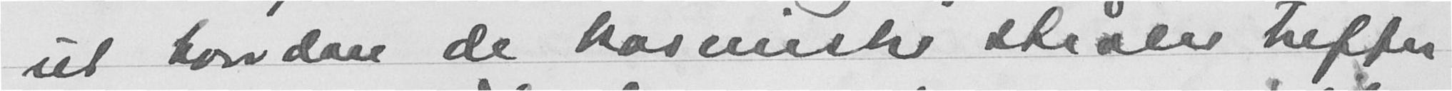ut hvorden de kosmiske stråler treffer
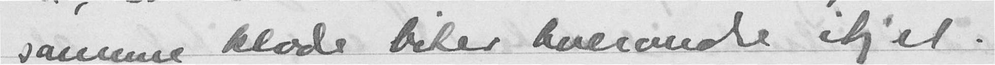samme klode biter hverandre ihjel.
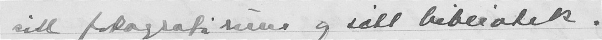sitt fotografirum og sitt bibliotek.
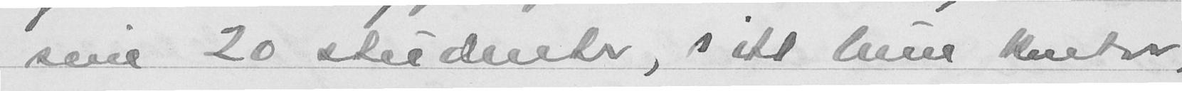sine 20 studenter, sitt lune kontor,
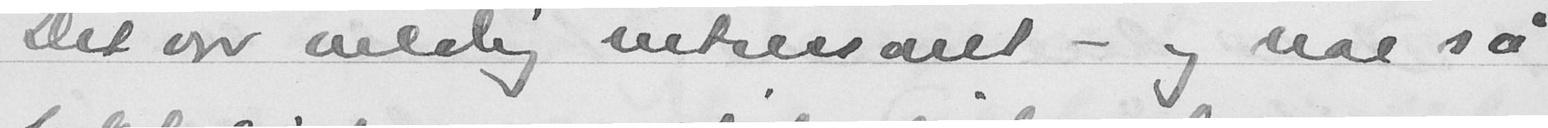Det var veldig intressant - og noe så
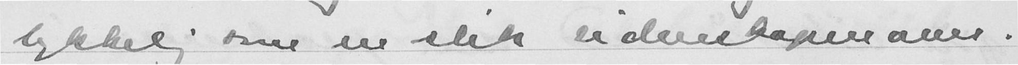lykkelig som en slik videnskapsmann.
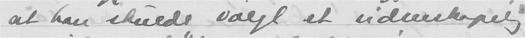at han skulde valgt et videnskapelig
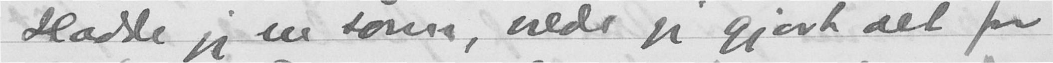Hadde jeg en sønn, vilde jeg gjort alt for
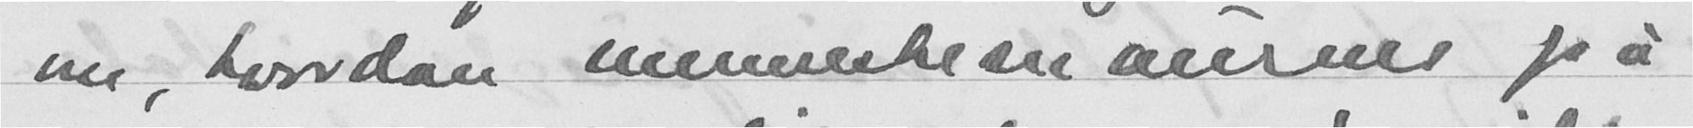om, hvordan menneskene sner på
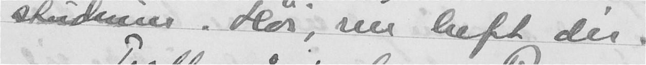studium. Hos, ren left des,
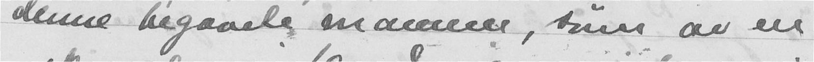denne begavete mannen, sønn av en
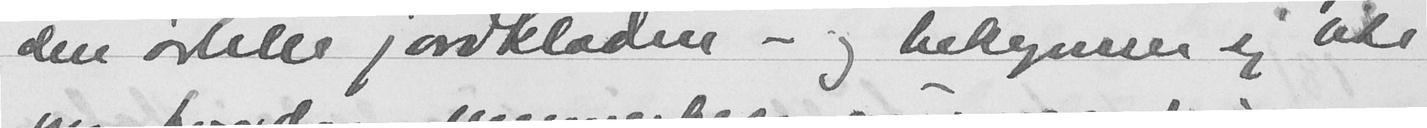den ørlille jordkloden - og bekymrer sig lite
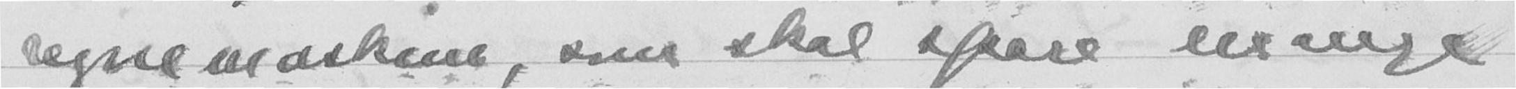regnemaskine, som skal spare mange
